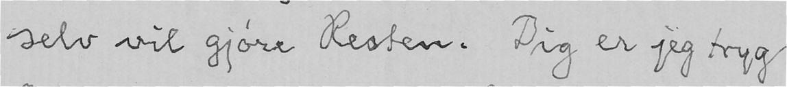selv vil gjøre Resten. Dig er jeg tryg
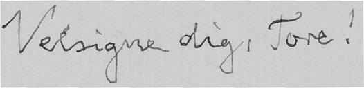Velsigne dig, Tore!
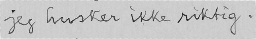jeg husker ikke riktig.
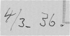4/3. 36.
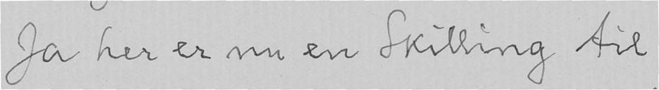Ja her er nu en Skilling til
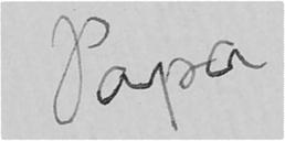Papa
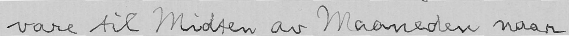vare til Midten av Maaneden naar
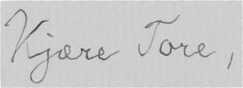Kjære Tore,
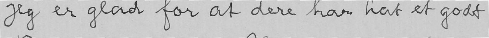jeg er glad for at dere har hat et godt
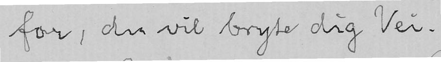for, du vil bryte dig Vei.
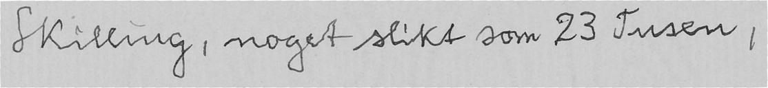Skilling, noget slikt som 23 Tusen,
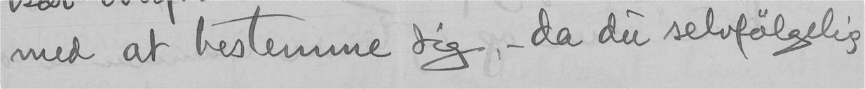med at bestemme dig, - da du selvfølgelig
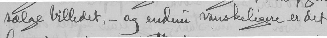sælge billedet, - og endnu vanskeligere er det
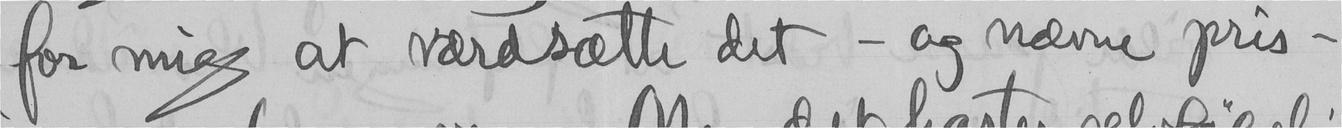for mig at værdsætte det - og nævne pris -
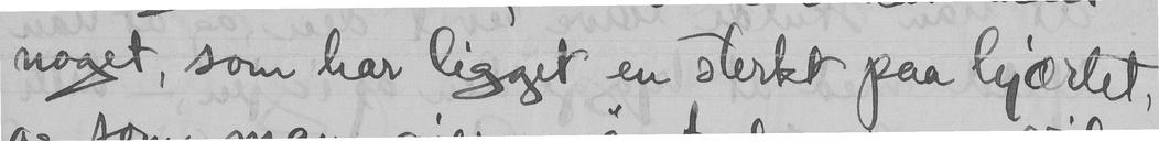noget, som har ligget en sterkt paa hjærtet,
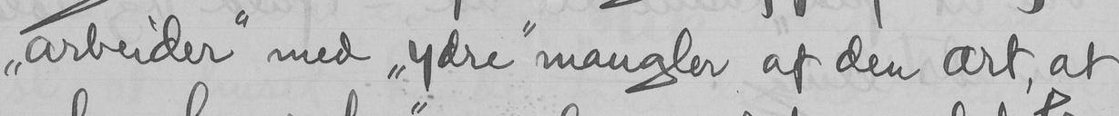"arbeider" med "ydre" mangler af den art, at
